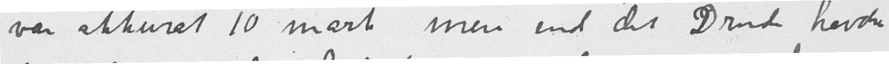var akkurat 10 mark mere end det Drude havde
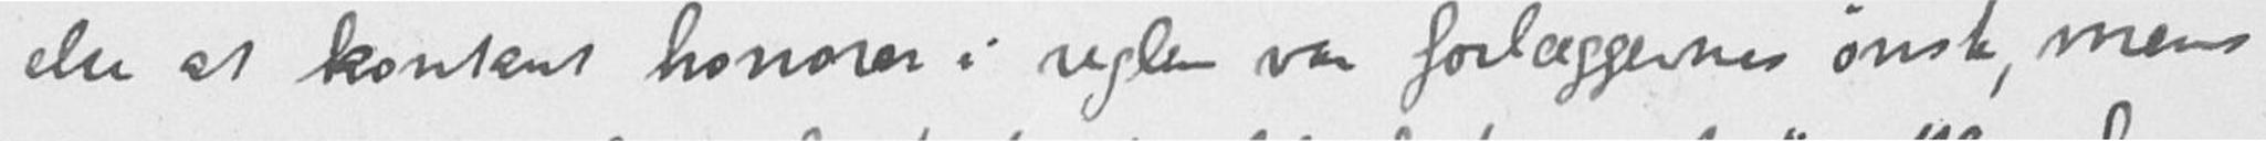else at kontant honorar i reglen var forlæggernes ønske, mens
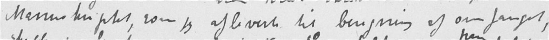Manuskriptet, som jeg afleverte til beregning af omfanget,
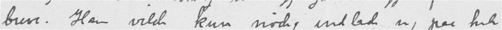breve. Han vilde kun nødig indlade sig paa hele
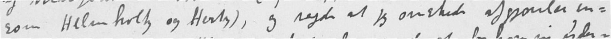som Helmholtz og Hertz), og sagde at jeg ønskede afgjørelse in-
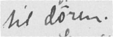til døren.
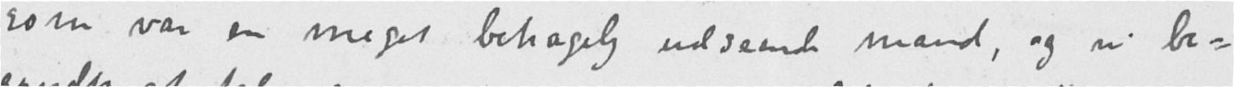som var en meget behagelig udseende mand, og vi be-
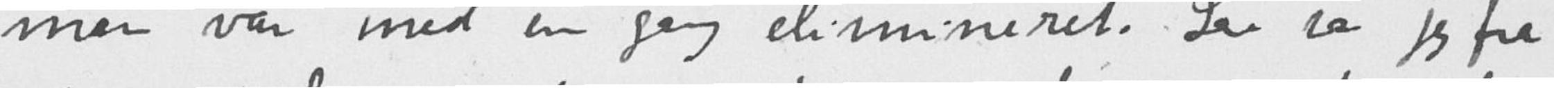man var med en gang elimineret. Saa sa jeg fra
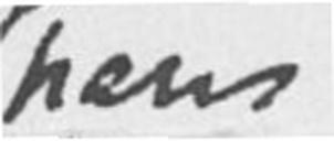hans
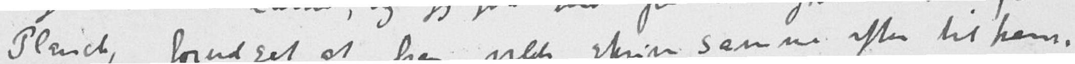Planck, forudsat at han vilde skrive samme aften til ham.
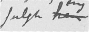fulgte ham
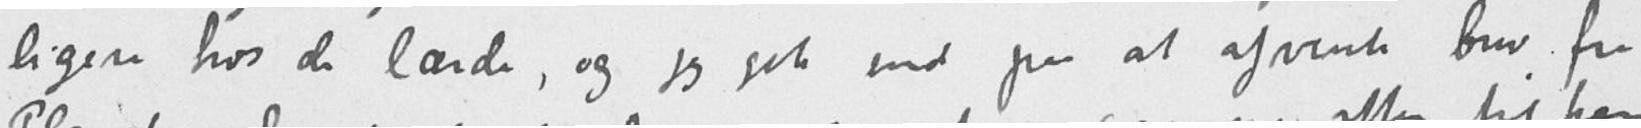ligere hos de lærde, og jeg gik ind paa at afvente brev fra
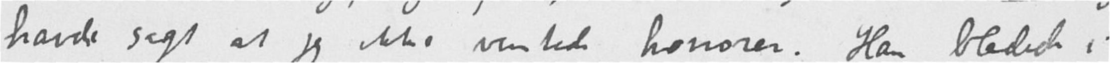havde sagt at jeg ikke ventede honorar. Han bladede i
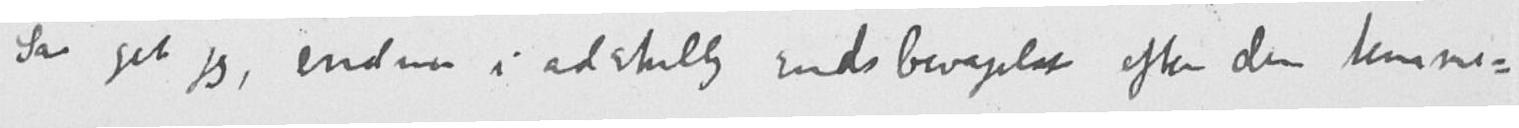Saa gik jeg, endnu i adskillig sindsbevægelse efter den temme-
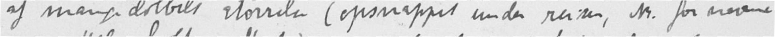af mangedobbelt størrelse (opsnappet under reisen, M. for navne
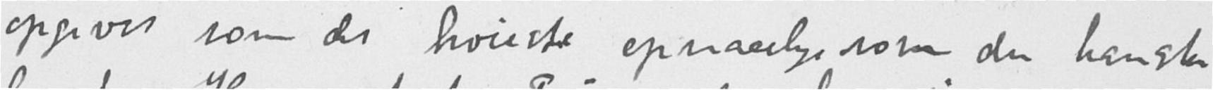opgivet som det høieste opnaaelige som du kanske
